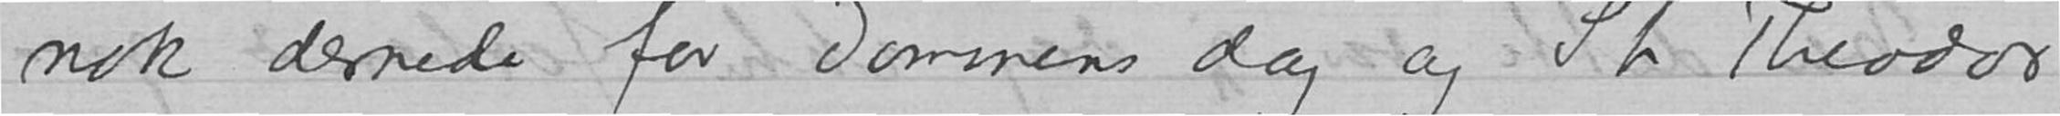nok dernede for Dommens dag og St Theodor
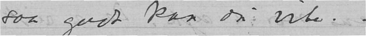saa godt kan du vite. -
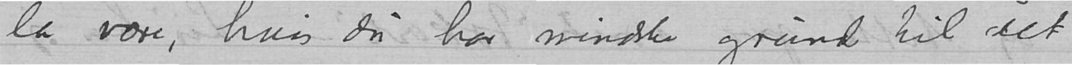la være, hvis du har mindste grund til det
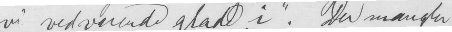vi vedvarende glade i. Der mangler
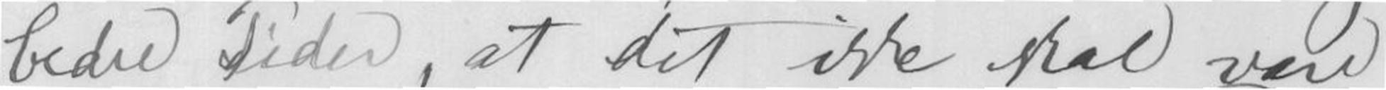bedre Tider, at det ikke skal vare
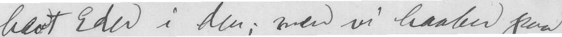havt Eder i den; men vi haaber paa
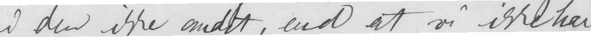i den ikke andet, end at vi ikke har
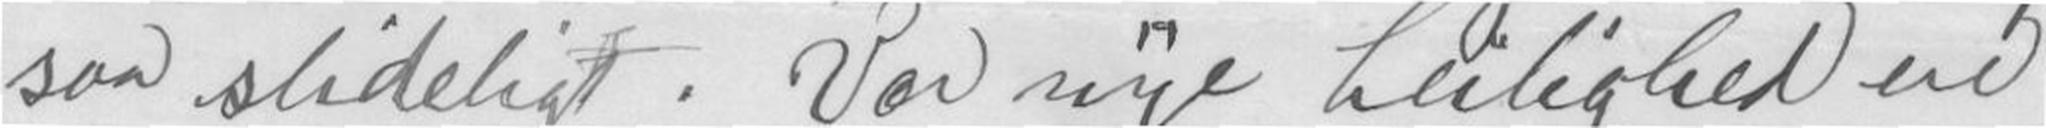som slideligt. Vor nye Leilighed ere
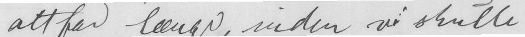alt for længe, inden vi skulle
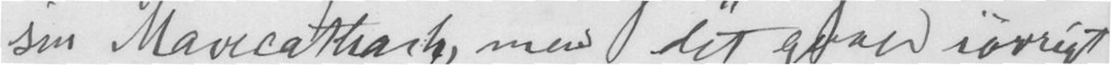sin Mavecatharh, men det gaar iøvrigt
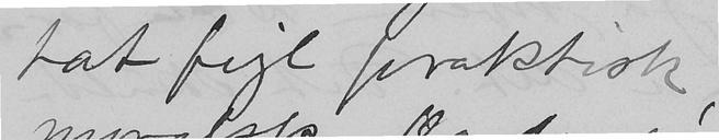tat fejl praktisk,
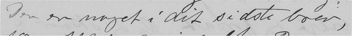Der er noget i dit sidste brev,
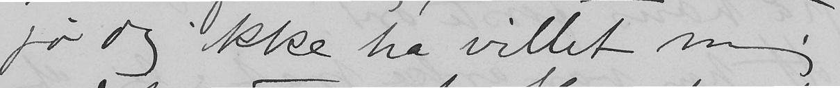jo dog ikke ha villet mig
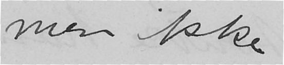men ikke
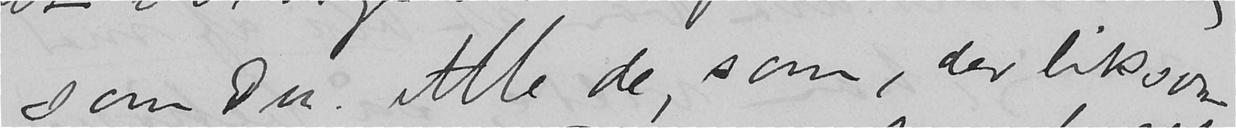som Du. Alle de, som, der liksom
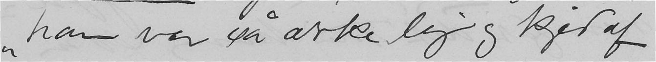"han var så ærke lej og kjed af
